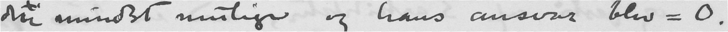det mindst mulige og hans ansvar blev = 0.
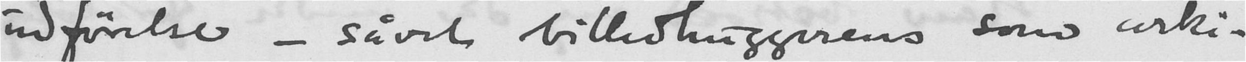udførelse - såvel billedhuggerens som arki-
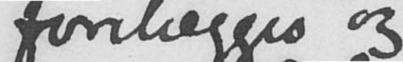forelægges og
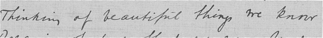Thinking of beautiful things we know
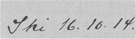Ski 16.10.14.
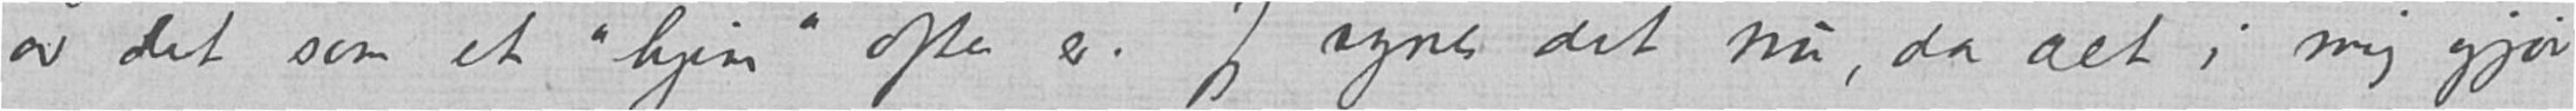av det som et "hjem" ofte er. Jeg synes det nu, da alt i mig gjør
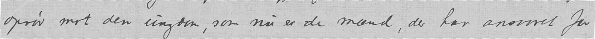oprør mot den ungdom, som nu er de mænd der han ansvaret for
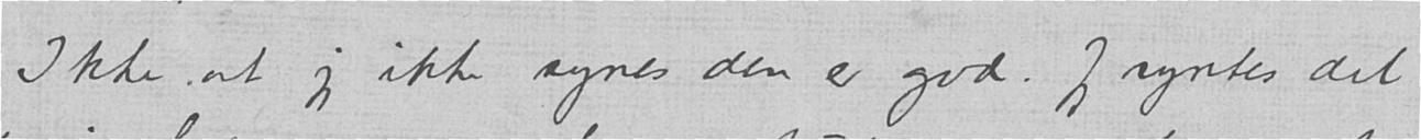Ikke at jeg ikke synes den er god. Jeg syntes det
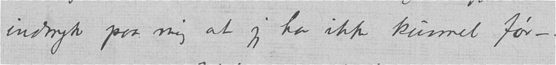indtryk paa mig at jeg har ikke kunnet før –.
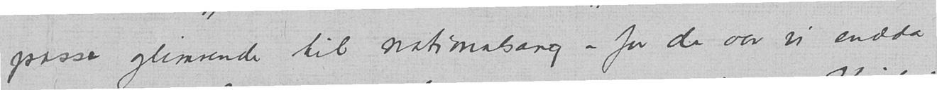passe glimrende til nationalsang – for de aar vi endda
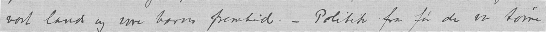vort lands og vore barns fremtid. - Politik fra før de var tørre
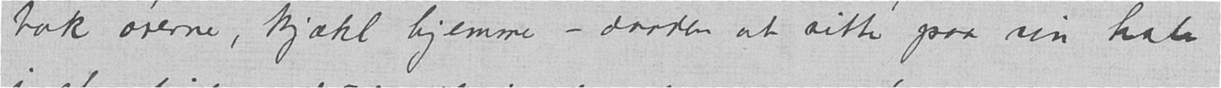bak ørerne, kjækl hjemme – daaden at sitte paa sin hale
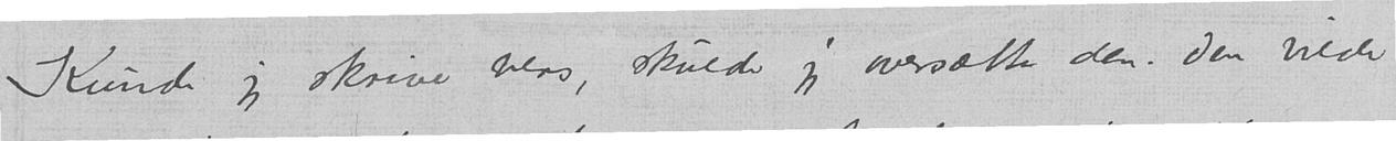- Kunde jeg skrive vers, skulde jeg oversætte den. Den vilde
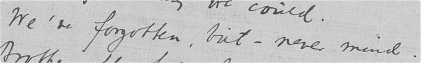We're forgotten, but – never mind.
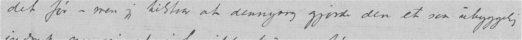det før – men jeg tilstaar at dennegang gjorde den et saa uhyggelig
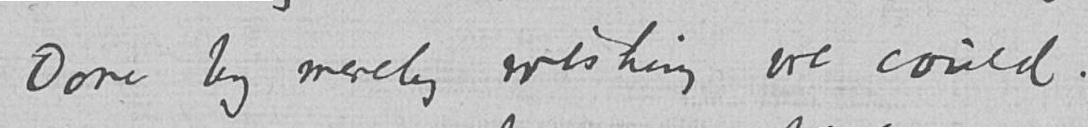Done by merely wishing we could.
